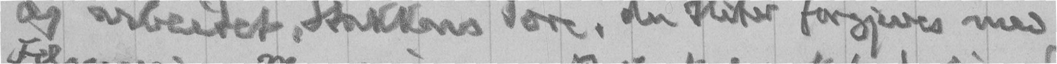og arbeidet. Stakkars Tore, du sliter forgjeves med
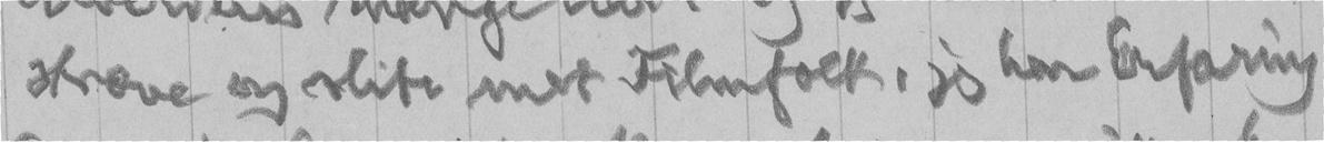stræve og slite med Filmfolk, jeg har Erfaring
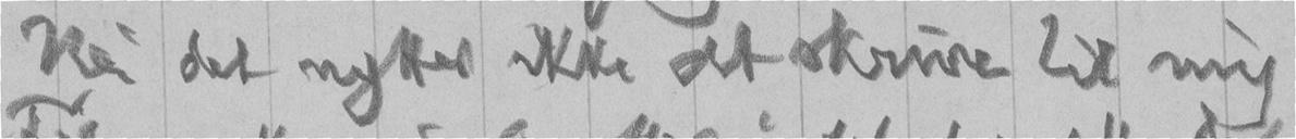Nei det nytter ikke at skrive til mig
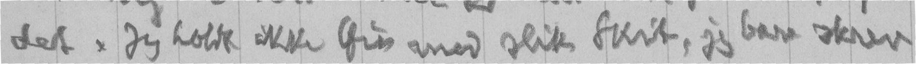det. Jeg holdt ikke Øie med slik Skit, jeg bare skrev
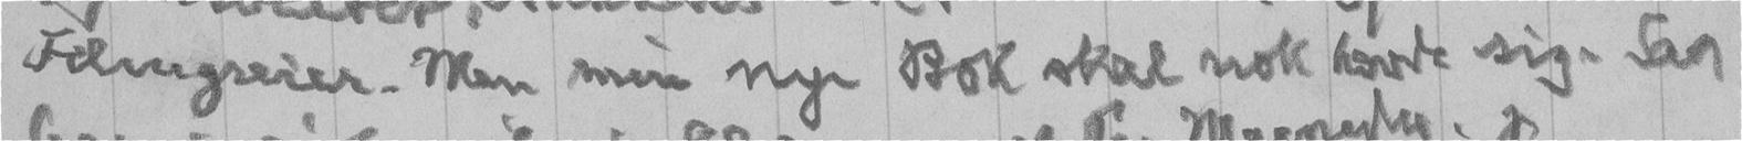Filmgreier. Men min nye Bok skal nok hævde sig. Saa
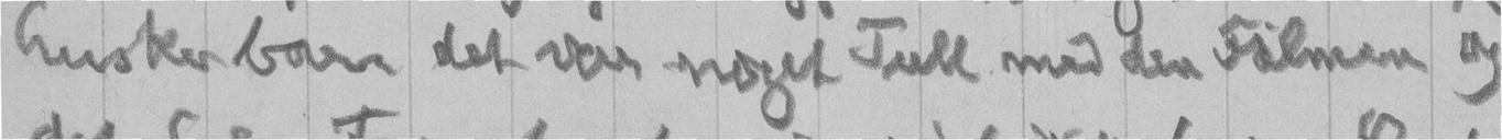husker bare det var noget Tull med den Filmen og
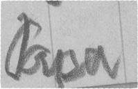Papa
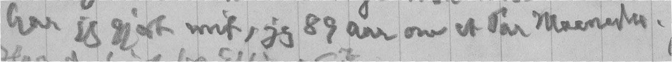har jeg gjort mit, jeg 89 Aar om et Par Maaneder.
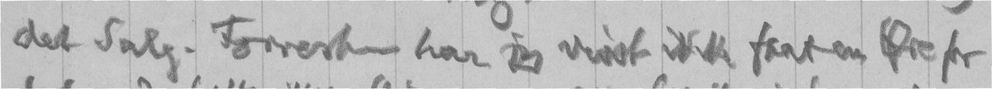det Salg. Forresten har jeg visst ikke faat en Øre for
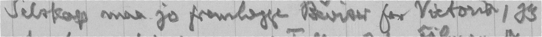Selskap maa jo fremlægge Beviser for Victoria, jeg
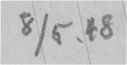8/5. 48
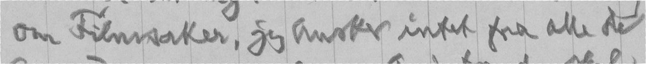om Filmsaker, jeg husker intet fra alle de
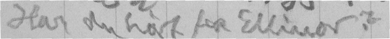Har du hørt fra Ellinor?
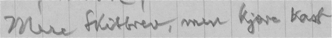Mere Skitbrev, men kjære kast
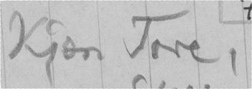Kjære Tore,
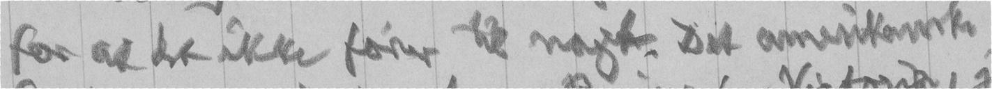for at det ikke fører til noget. Det amerikanske
